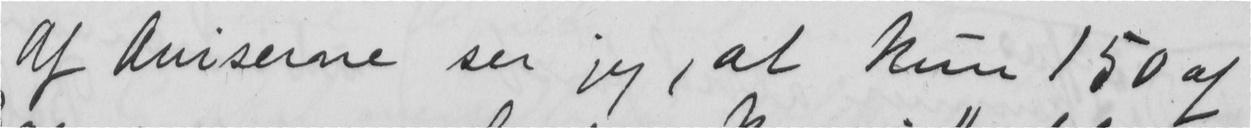Af aviserne ser jeg, at kun 150 af
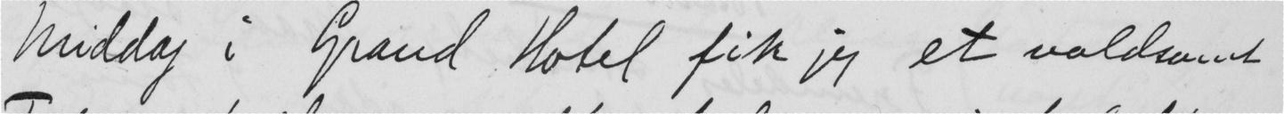Middag i Grand Hotel fik jeg et voldsomt
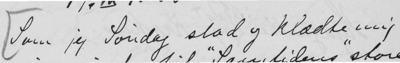Som jeg Søndag stod og klædte mig
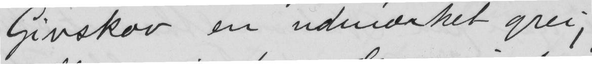Givskov en udmærket grei,
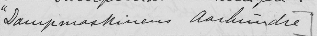"Dampmaskinens Aarhundre"
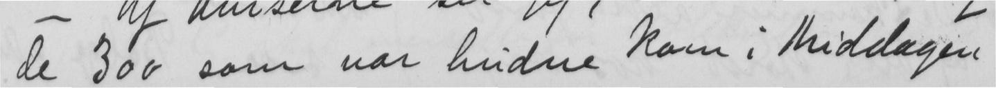de 300 som var budne kom i Middagen
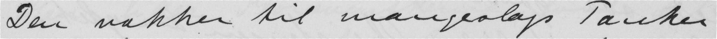Den vækker til mangeslags Tanker
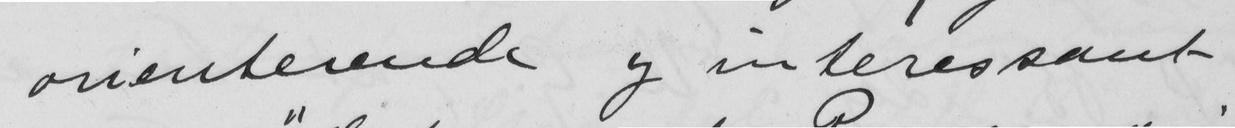orienterende og interessant
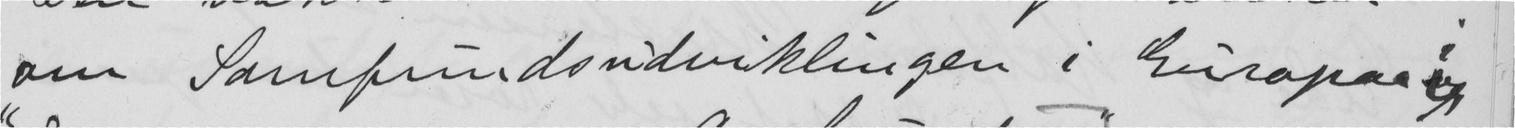om Samfrindsudviklingen i Europa i
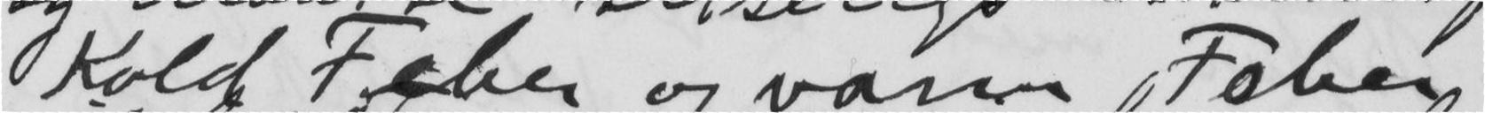Kold Fober og varm Feber
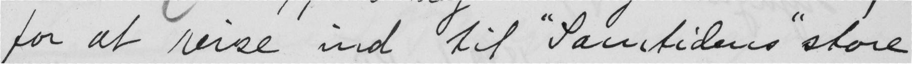for at reise ind til "Samtidens" store
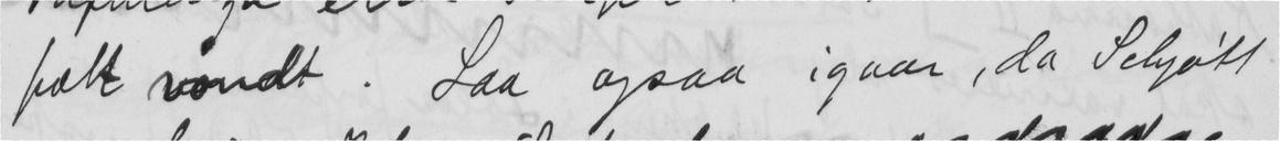fælt vondt. Laa ogsaa igaar, da Schjøtt
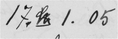17. 1.05
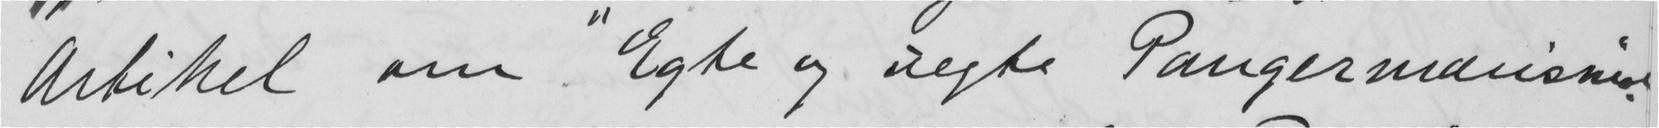Artikel om "Egte og uegte Pangermansnen.
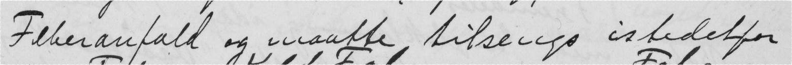Feberanfald og maatte tilsengs istedetfor
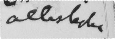allesteder
